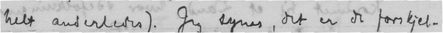helt anderledes). Jeg synes, det er de forskjel-
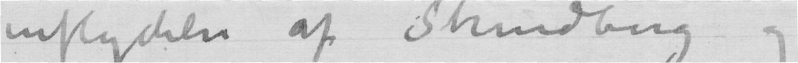inflydelse af Strindberg og
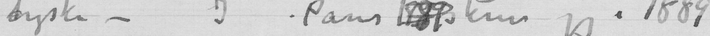tyske – I Paris 1889skrev jeg i 1889
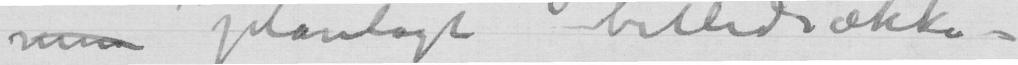min planlagt billedrække –
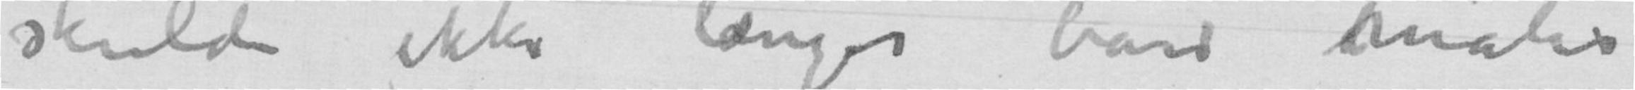skulde ikke længer bare males
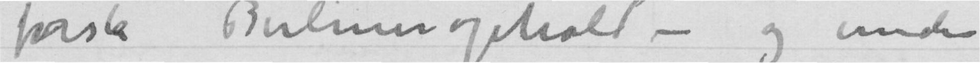første Berlinophold – og under
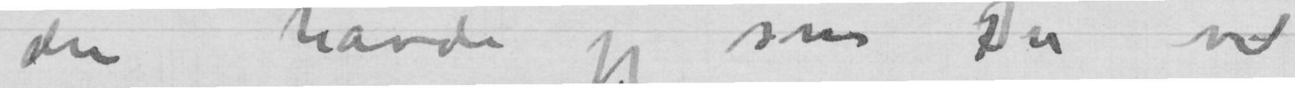den havde jeg som Du vet
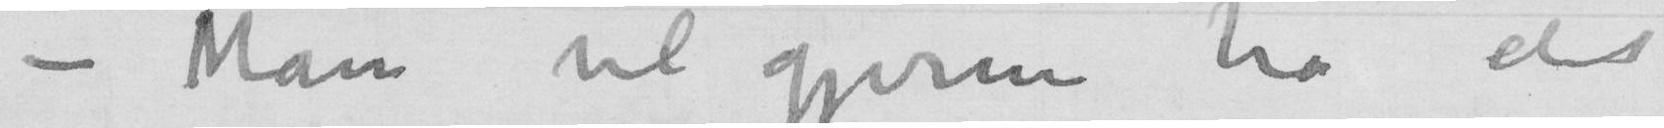– Man vil gjerne ha det
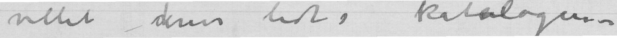villet skrive lidt i katalogen –
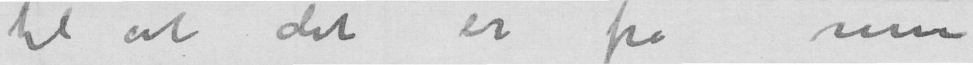til at det er fra min
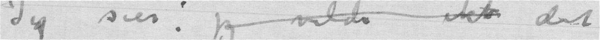Jeg sier: jeg vilde ikk det
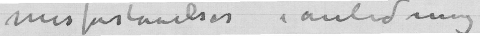misforståelser i anledning
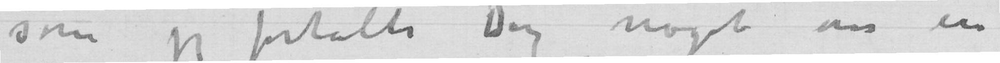som jeg fortalte Dig noget om en
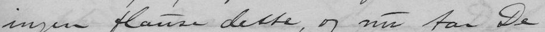ingen flause dette, og nu tar De
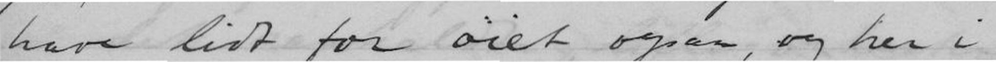have lidt for øiet ogsaa, og her i
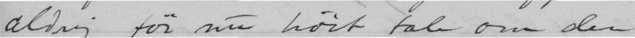aldrig før nu hørt tale om den
